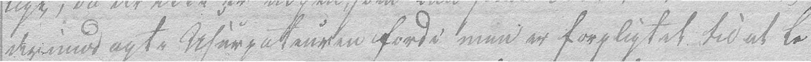derimod agte Usurpateuren fordi man er forpligtet til at le-
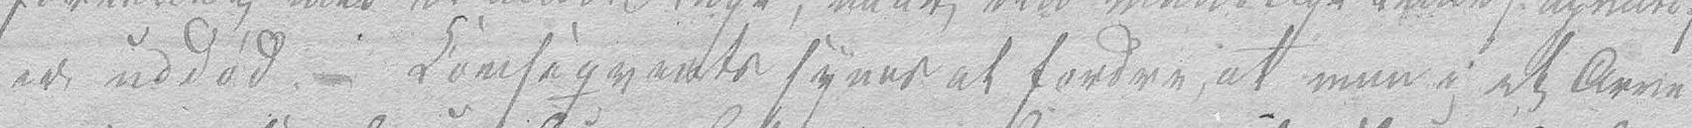er uddød. – Conseqvents synes at fordre, at man i et Arve-
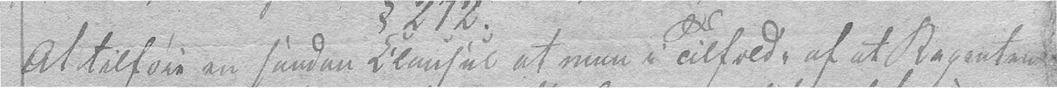At tilføie en saadan Clausul at man i Tilfælde af at Regenten
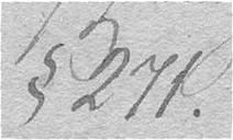§ 271.
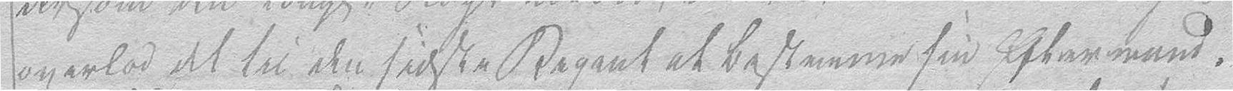overlod det til den sidste Regent at bestemme sin Eftermand.
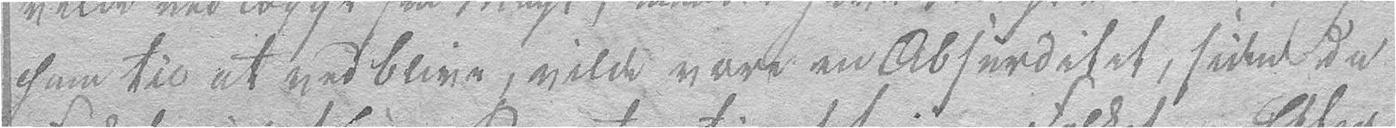han til at vedblive, vilde være en Absurditet, siden da
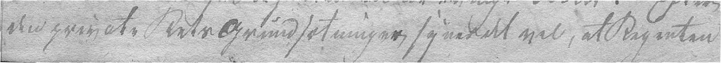den private Rets Grundsætninger synes det vel, at Regenten
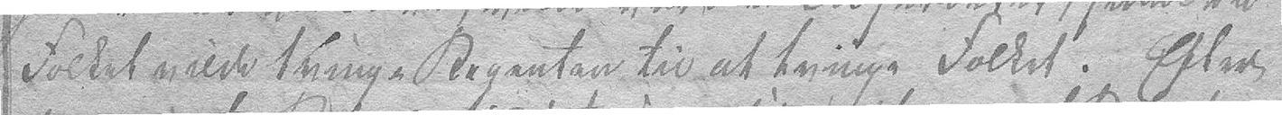Folket vilde tvinge Regenten til at tvinge Folket. Efter
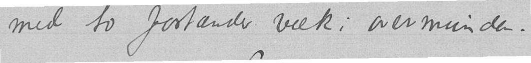med to fortænder væk i overmunden.
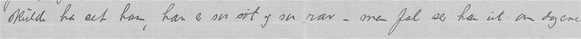skulde ha set ham, han er saa søt og saa rar – men fæl ser han ut om dagene
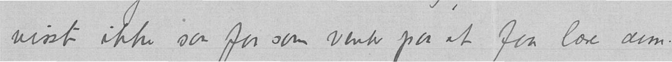visst ikke saa faa som venter paa at faa læse dem.
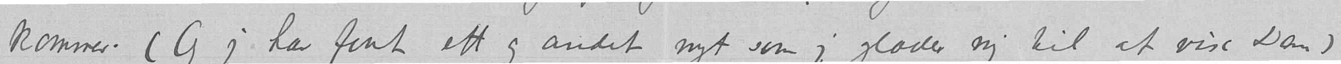kommer. (Og jeg har faat ett og andet nyt som jeg glæder mig til at vise Dem)
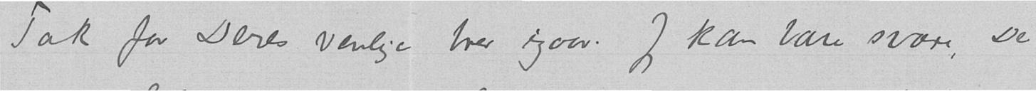Tak for Deres venlige brev igaar. Jeg kan bare svare, De
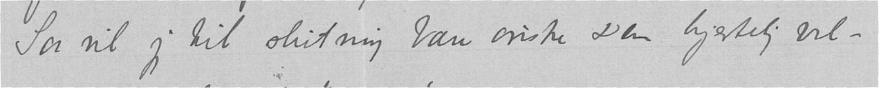Saa vil jeg til slutning bare ønske Dem hjertelig vel-
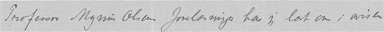Professor Magnus Olsens forelæsninger har jeg læst om i avisen
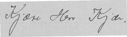Kjære Herr Kjær,
